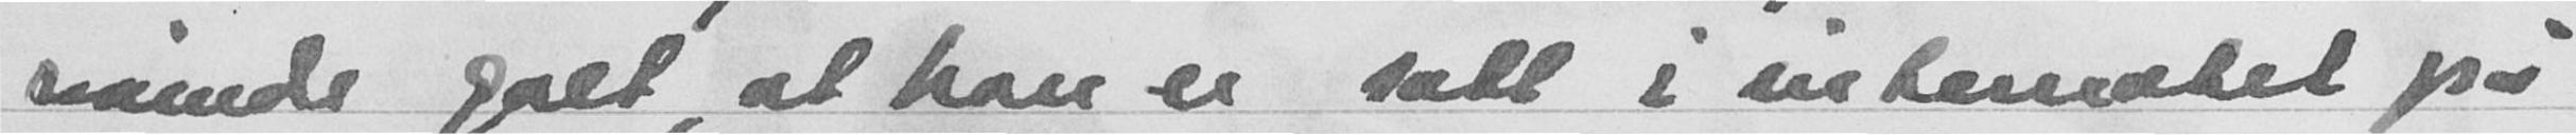rande galt, at han er satt i internatet på
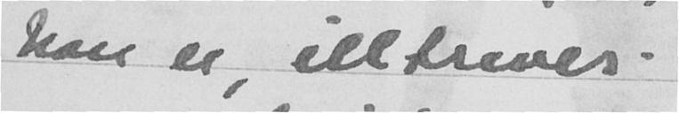han er, illtrives.
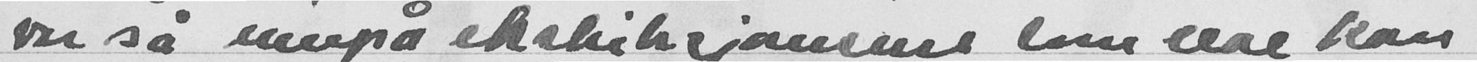var så innpå ekskeksjansens som noe kan
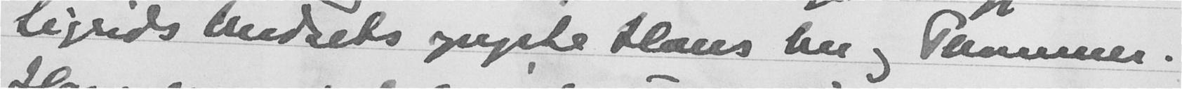Sigrids Undsets yngste Hans her og Thommes.
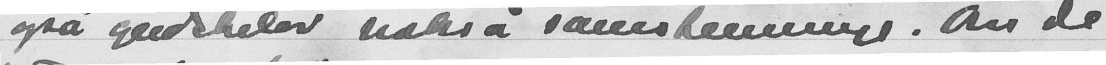også gudskelov nokså samstemmige. Om de
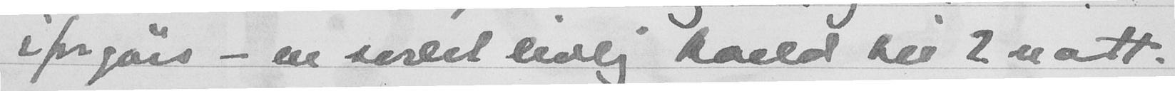iforgårs - en svalet tidlig kveld til 2 natt.
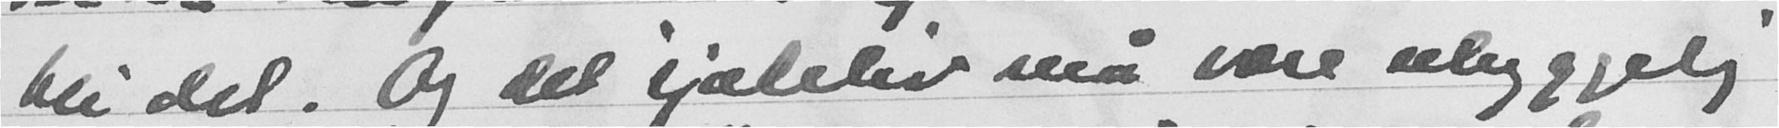bli det. Og det sjæleliv må være uhyggelig
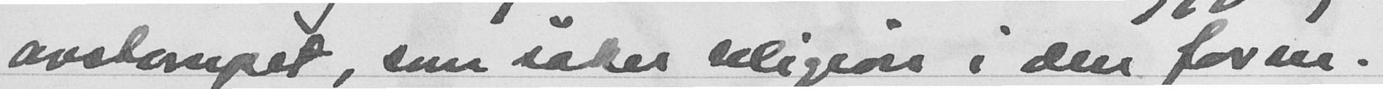avstompet, som søker religion i den form.
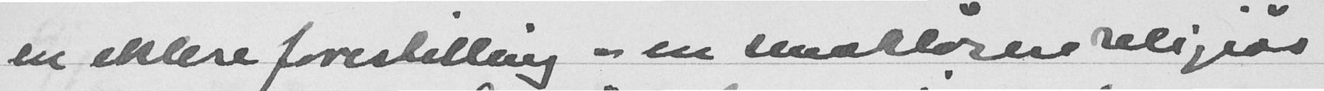en eklere forestilling - en smakløsere religiøs
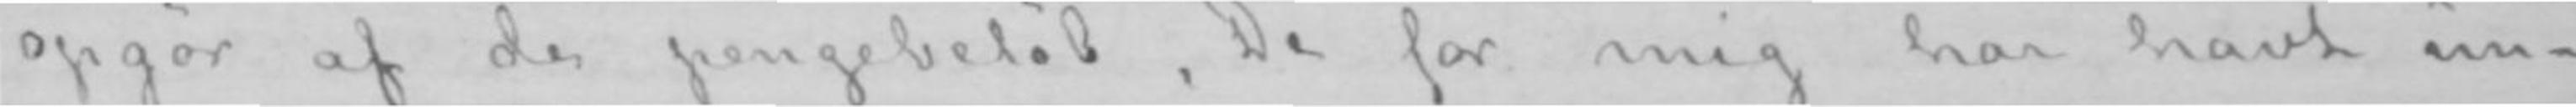opgør af de pengebeløb, De for mig har havt un-
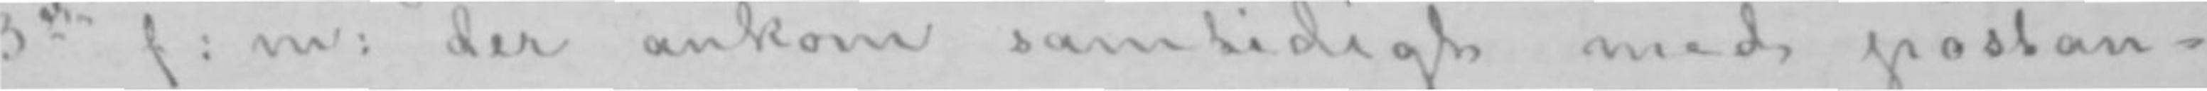af 3die f: m: der ankom samtidigt med postan-
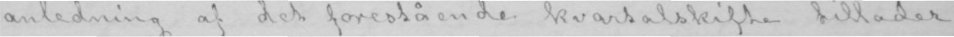anledning af det forestående kvartalskifte tillader
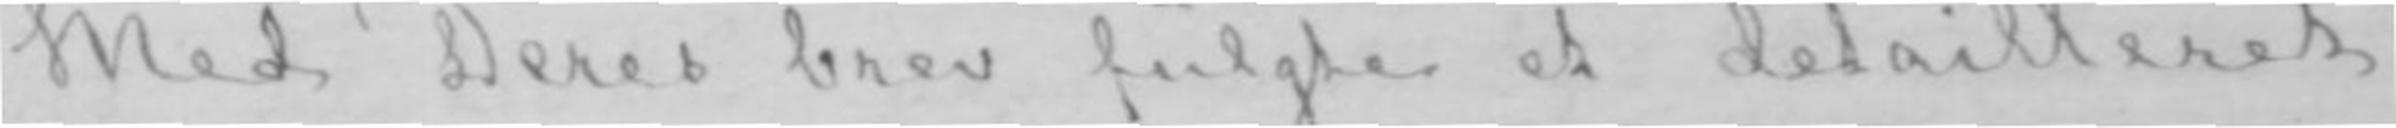Med Deres brev fulgte et detailleret
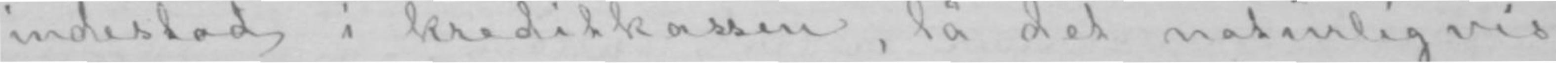indestod i kreditkassen, lå det naturligvis
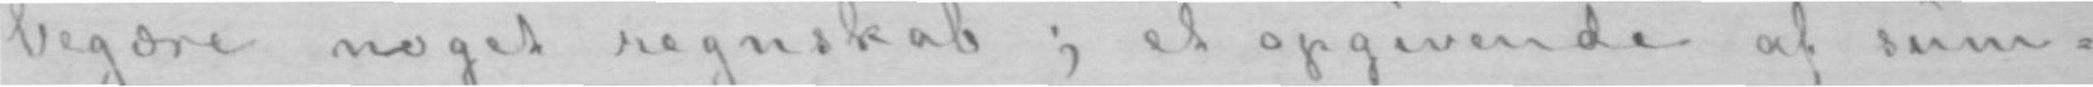begære noget regnskab; et opgivende af sum-
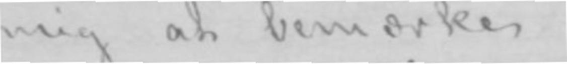mig at bemærke.
